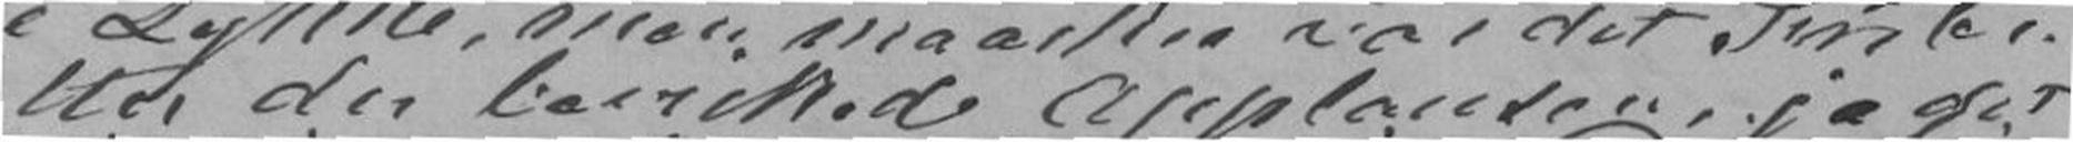letter der bevirkede applausen, ja det
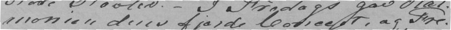monien dens fjerde Concert, og Fre-
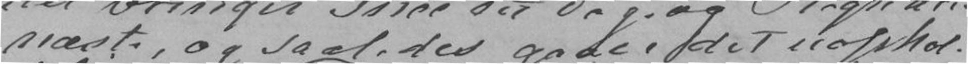næste, og saaledes gaaer det uophol-
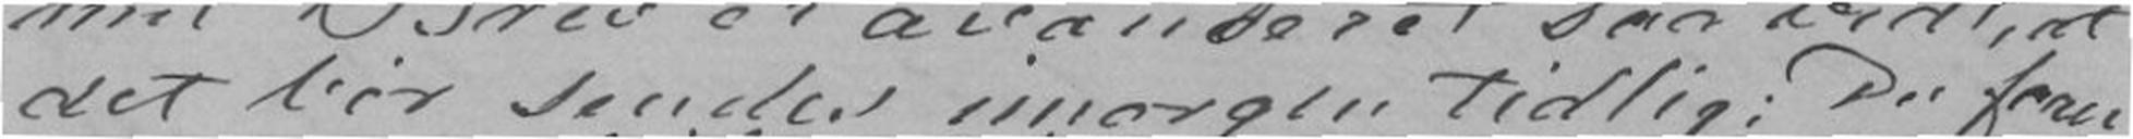det bør sendes imorgen tidlig; Du faaer
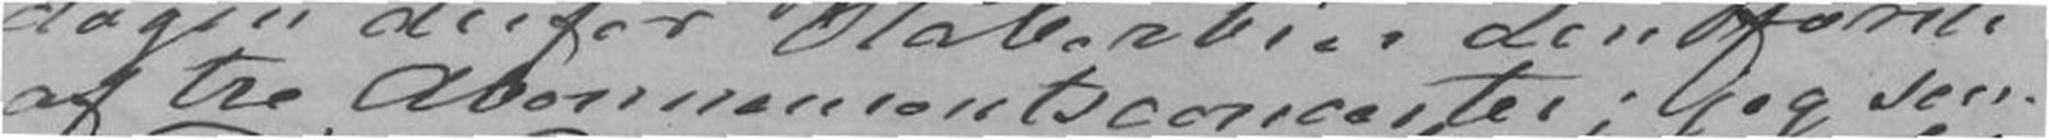af tre Abonnementsconcerter; jeg sen-
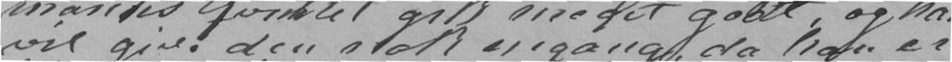vil give den nok engang, da han er
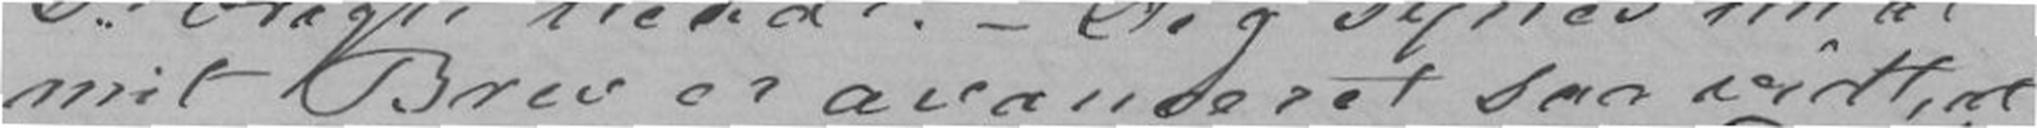mit Brev er avanteret saa vidt, at
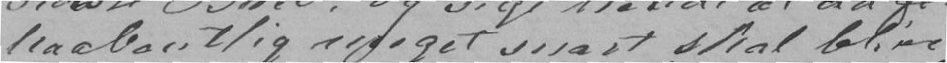haabentlig meget snart skal blive
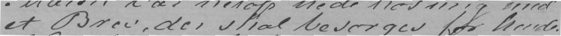et Brev, der skal besørges for hende.
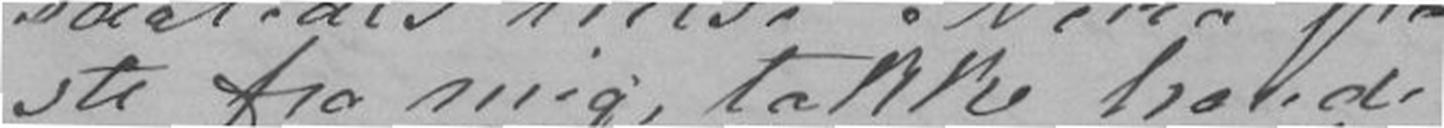ste fra mig, takke hende
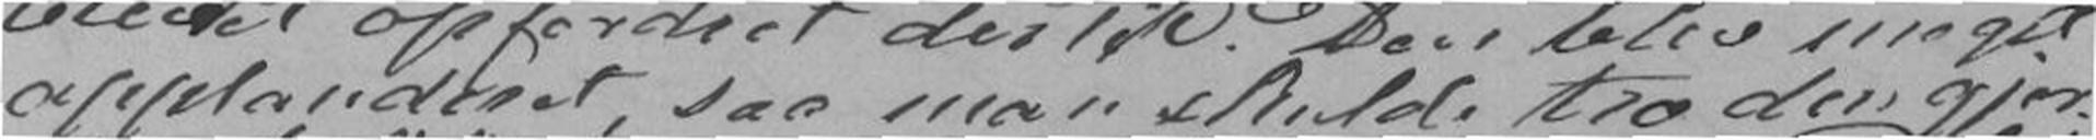applauderet, saa man skulde tro den gjor-
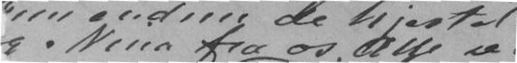Nina fra os Alle ved
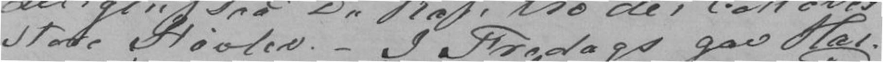store Støvler. - I Fredags gav Her-
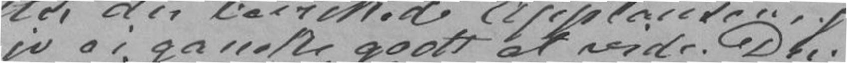jo ei ganske godt vide. Din
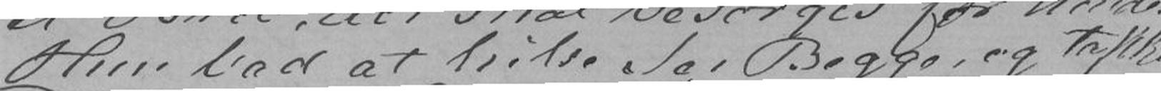Hun bad at hilse Jer Begge, og takke
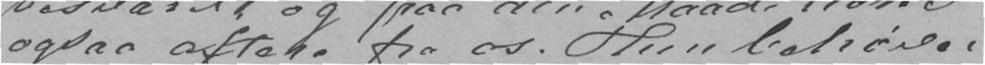ogsaa oftere fra os. Hun behøver
